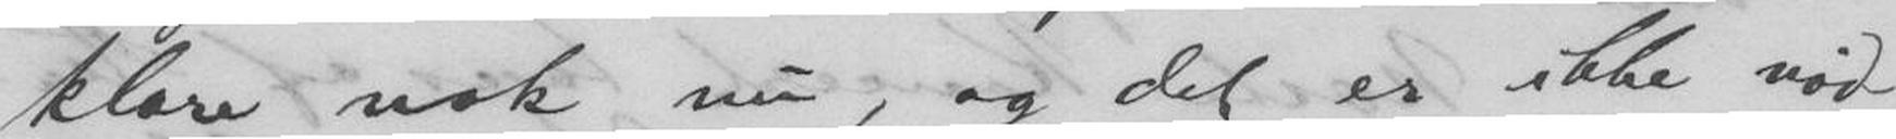klare nok nu, og det er ikke nød-
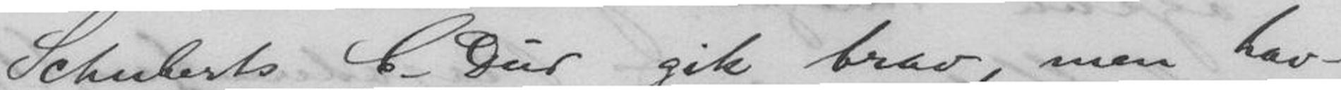Schubert C-Dur gik brav, men hav-
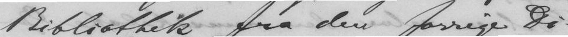Bibliothek fra den forrige Di-
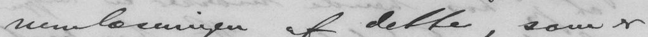nemlæsningen af dette, som er
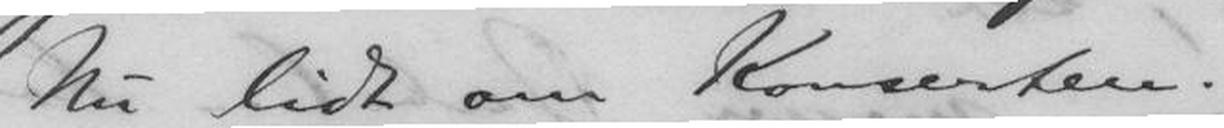Nu lidt om Konserten.
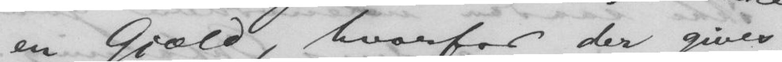en Gjæld, hvorfor der gives
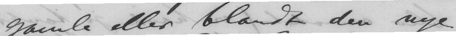gamle eller blandt den nye
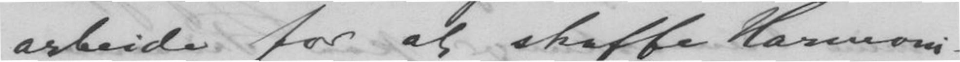arbeide for at skaffe Harmoni-
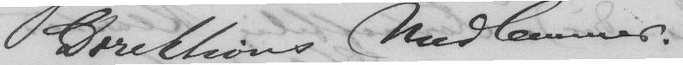Direktions Medlemmer.
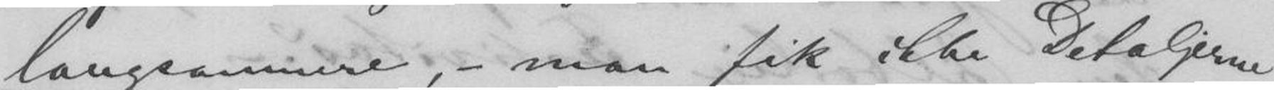langsommere, - man fik ikke Detaljerne
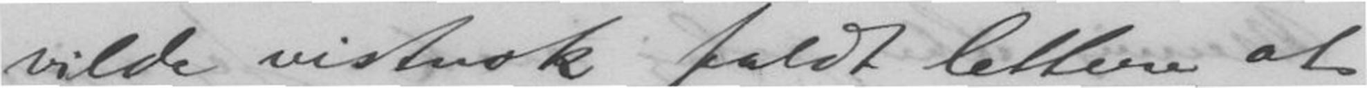vilde vistnok faldt lettere at
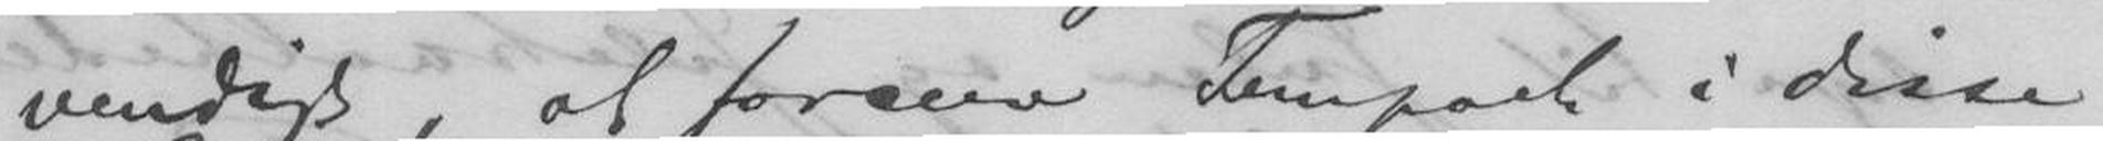vendigt, at forcere Tempoet i disse
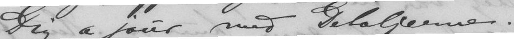Dig a jour med Detaljerne.
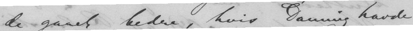de gaaet bedre, hvis Danning havde
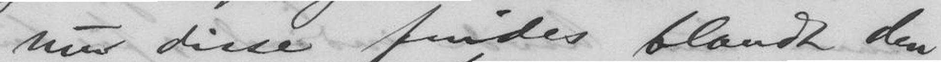nu disse findes blandt den
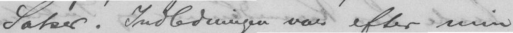Satser. Indledningen var efter min
